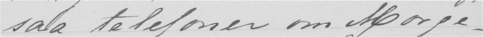saa telefoner om Morge-
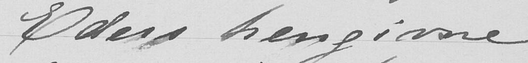Eders hengivne
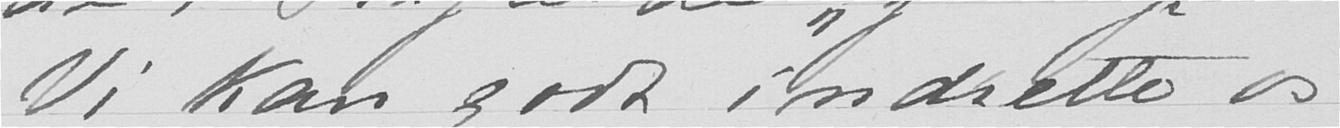Vi kan godt indrette os
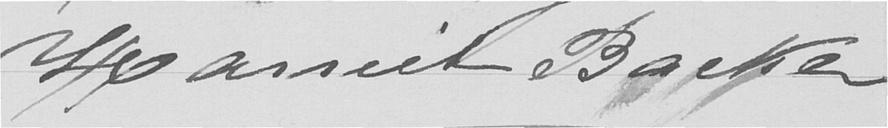Harriet Backer
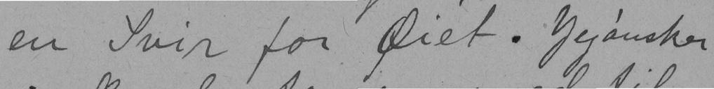en Svir for Øiet. Jeg ønsker
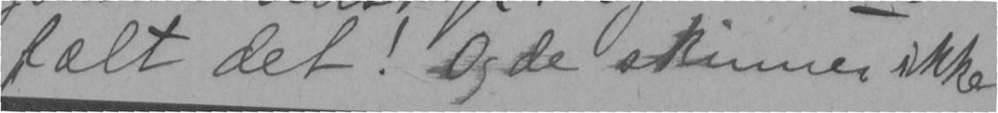fælt det! Og de skinner ikke
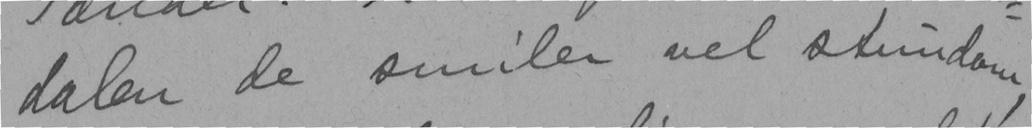dalen de smiler vel stundom,
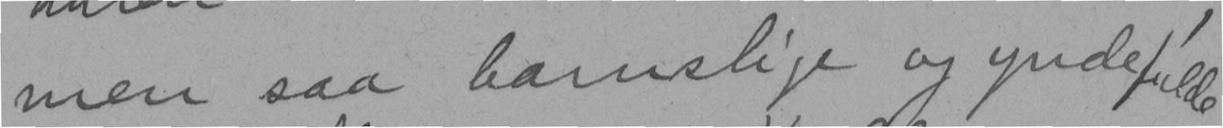men saa barnslige og yndefulde
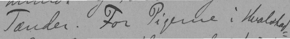Tænder. For Pigerne i Hvalstad-
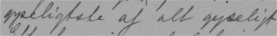gyseligtste af alt gyseligt
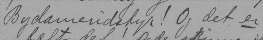Bydameudstur! Og det er
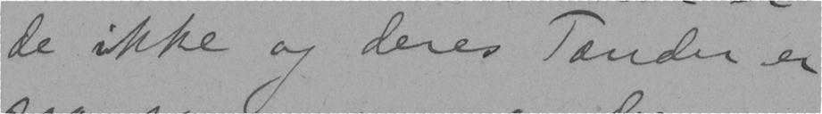de ikke og deres Tænder er
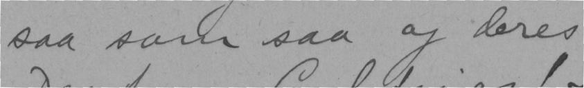saa som saa og deres
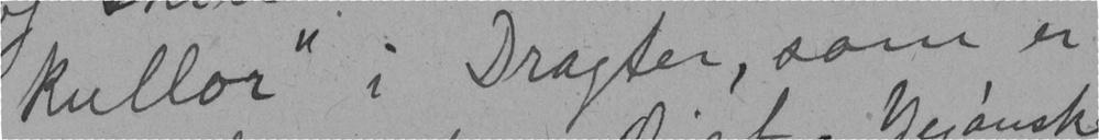kullor". Dragter, som er
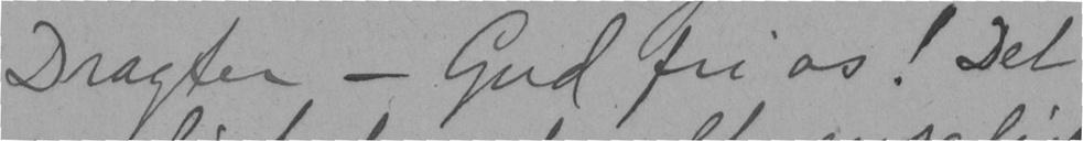Dragter - Gud fri os! Det
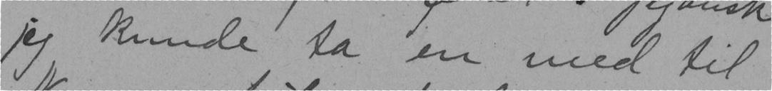jeg kunde ta en med til
